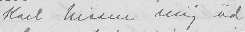Karl Nissen mig ud
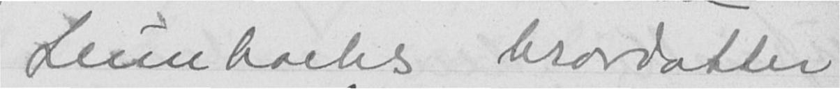Leuerbachs brordatter
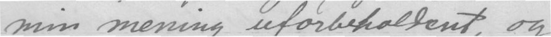min mening uforbeholdent, og
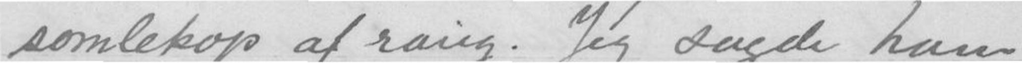somlekop af rang. Jeg sagde ham
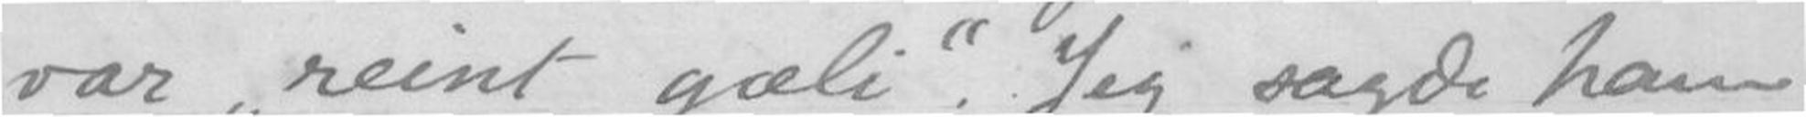var reint gæli Jeg sagde ham
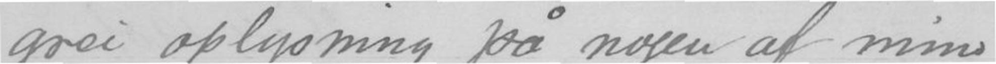grei oplysning på nogen af mine
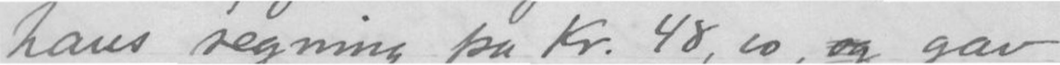hans regning på Kr. 48,10,og gav
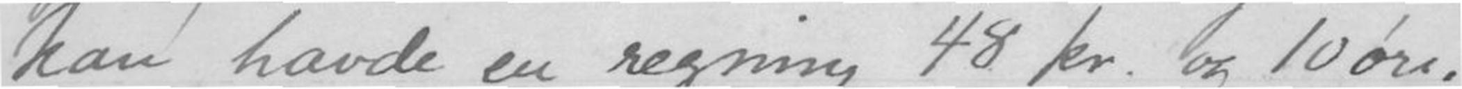han havde en regning 48 kr. og 10 øre.
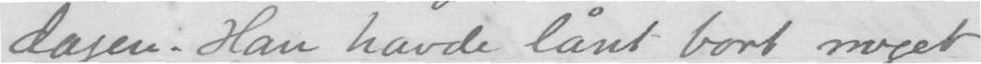dagen. Han havde lånt bort noget
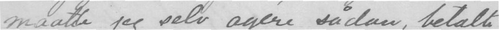maatte jeg selv agére sådan, betalte
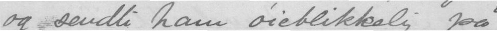og sendte ham øieblikkelig på
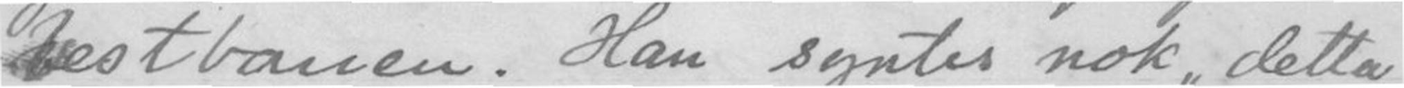Vestbanen. Han syntes nok "detta
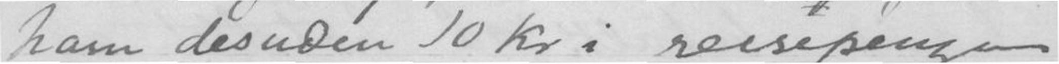ham desuden 10 kr i reisepenger
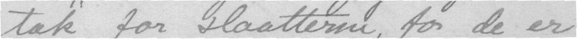tak for slaatterne, for de er
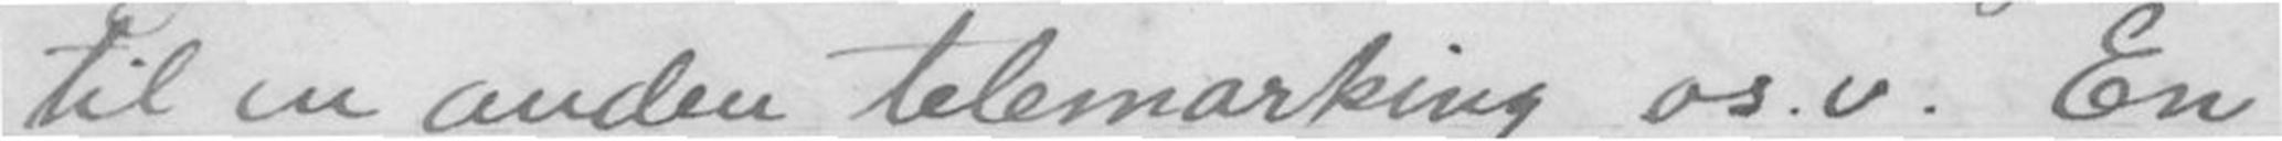til en anden telemarking os.v. En
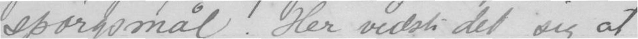spørgsmål. Her vidste det sig at
